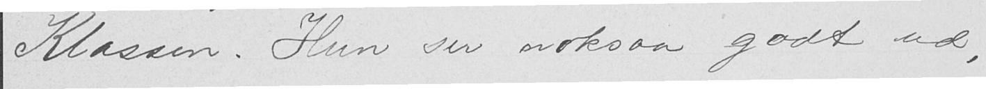Klassen. Hun ser noksaa godt ud,
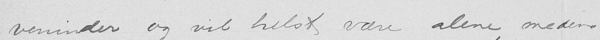veninder og vil helst være alene, medens
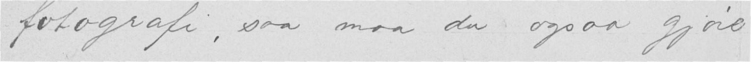fotografi, saa maa de ogsaa gjøre
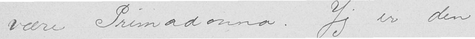være Primadonna. Jeg er den
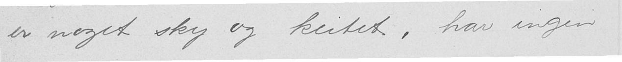er noget sky og keitet, har ingen
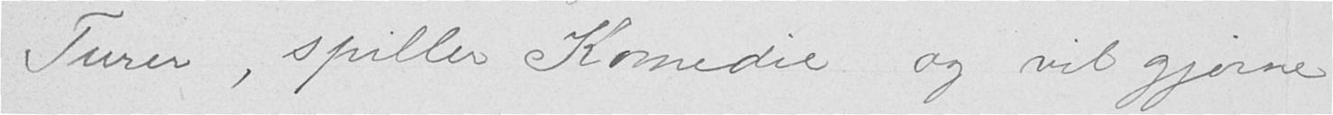Turer, spiller Komedie og vil gjerne
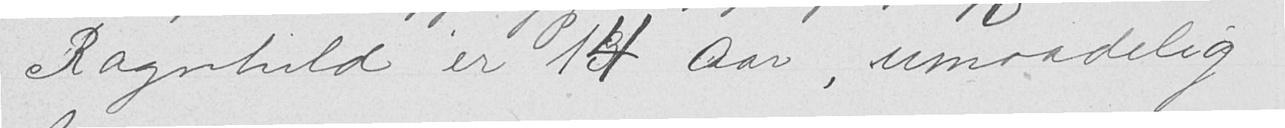Ragnhild er 14 Aar, umaadelig
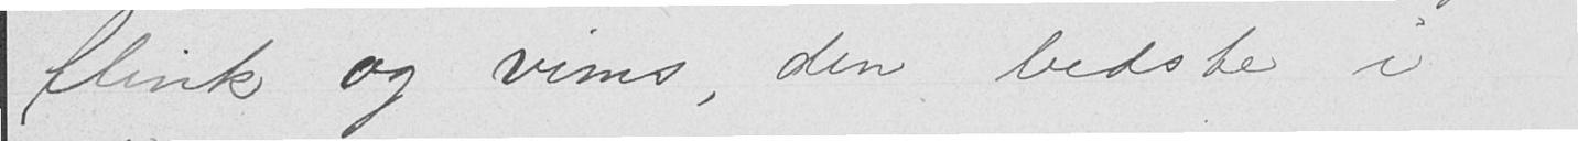flink og vims, den bedste
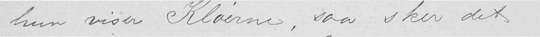hun viser Klørne, saa sker det
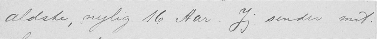ældste, nylig 16 Aar. Jeg sender mit
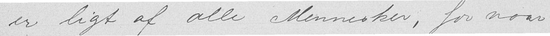er ligt af alle Mennesker, for naar
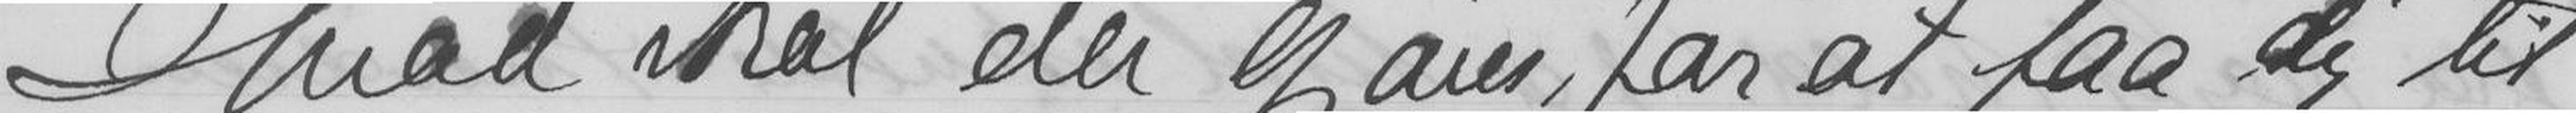Hvad skal der gjøres for at faa dig til
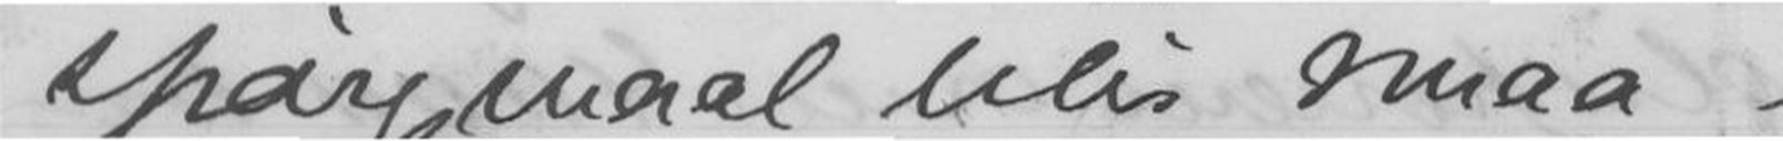spørgsmaal blir smaa!
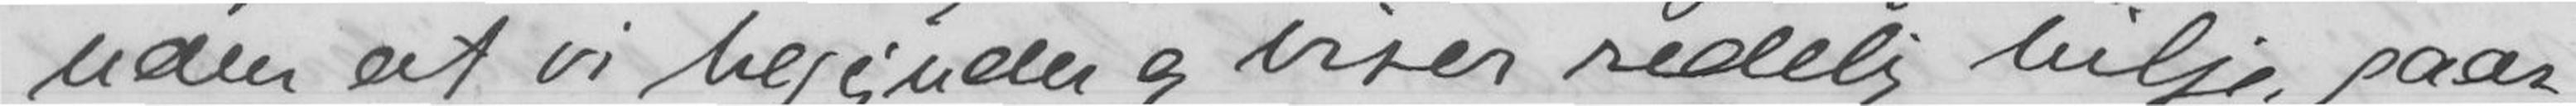uden at vi begynder og viser redelig vilje gaar
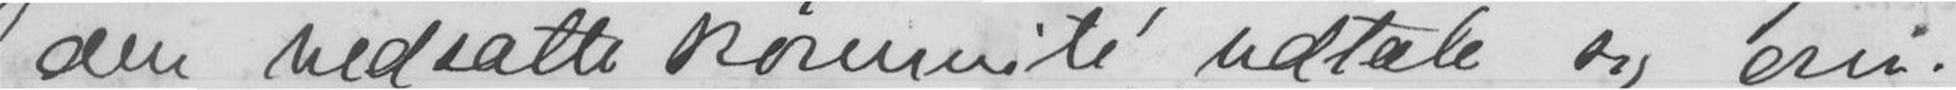den nedsatte kommitè udtale sig om.
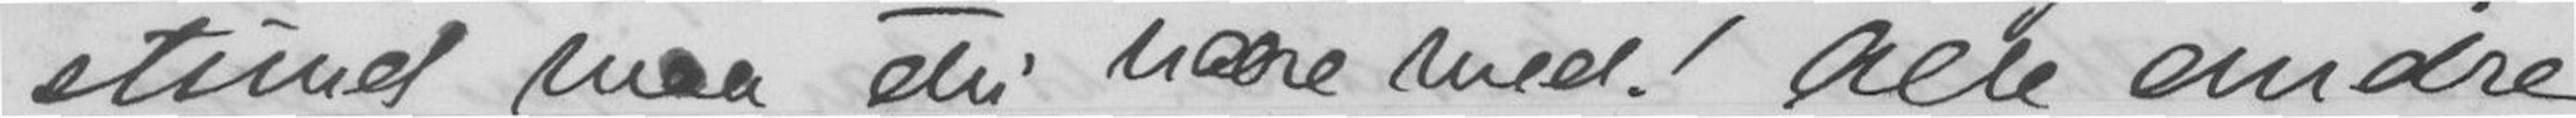stund maa du være med! Alle andre
